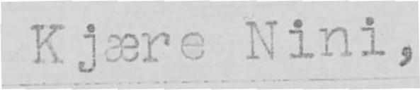Kjære Nini,
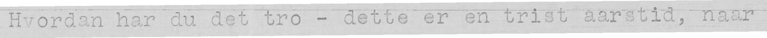Hvordan har du det tro – dette er en trist aarstid, naar
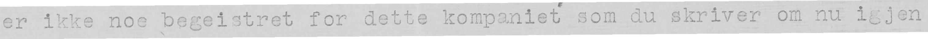er ikke noe begeistret for dette kompaniet som du skriver om nu igjen
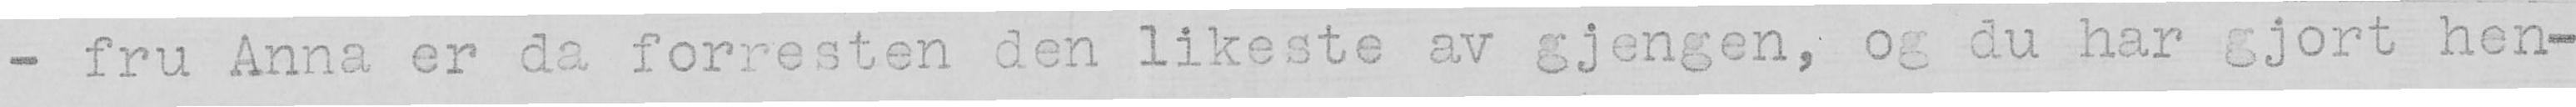– fru Anna er da forresten den likeste av gjengen, og du har gjort hen-
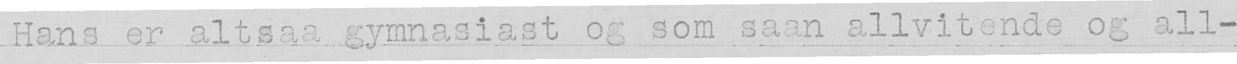Hans er altsaa gymnasiast og som saan allvitende og all-
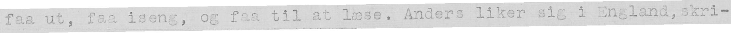faa ut, faa iseng, og faa til at læse. Anders liker seg i England, skri-
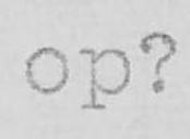op?
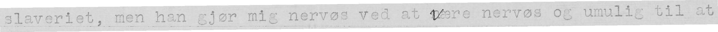slaveriet, men han gjør mig nervøs ved at være nervøs og umulig til at
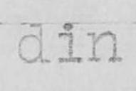din
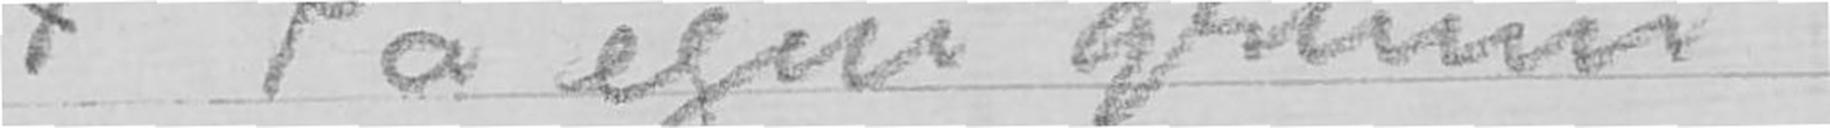«På egen grunn»
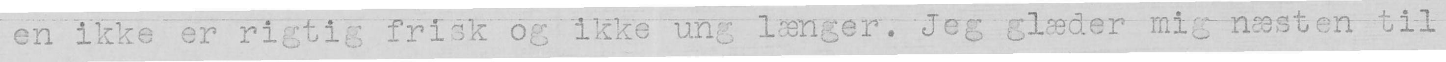en ikke er rigtig frisk og ikke ung længer. Jeg glæder mig næsten til
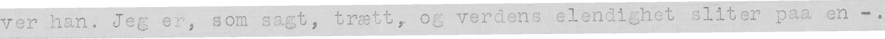ver han. Jeg er, som sagt, trætt, og verdens elendighet sliter paa en –.
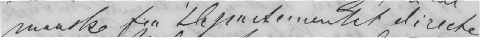maaske fra Departementet directe
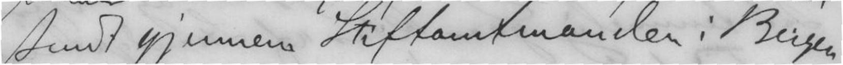sendt gjennem Stiftamtmanden i Bergen.
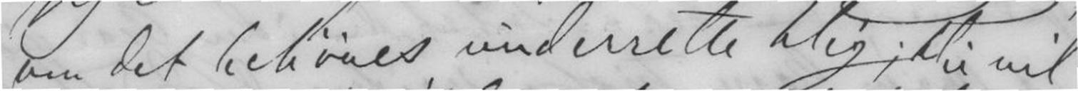om det behøves underrette Dig; Du vil
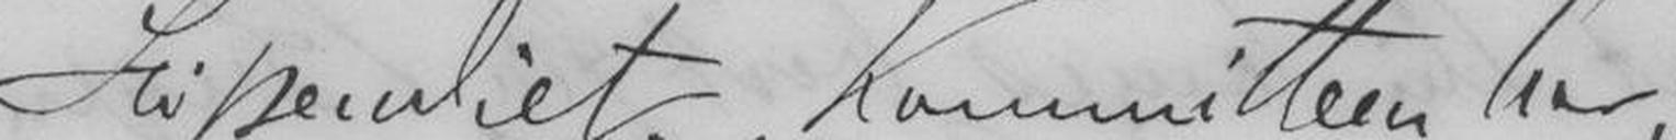Stipendiet, Kommitteen har
